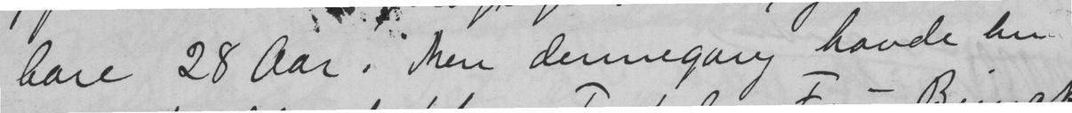bare 28 aar. Men dennegang havde hun
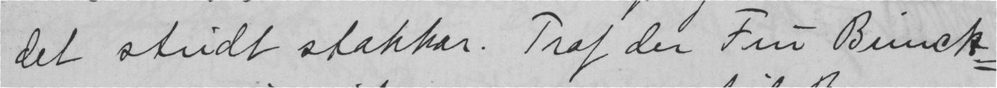det stridt stakkar. Traf der Fru Brinck-
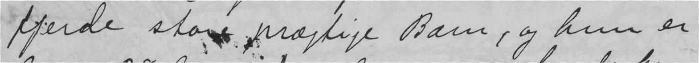fjerde store prægtige Barn, og hun er
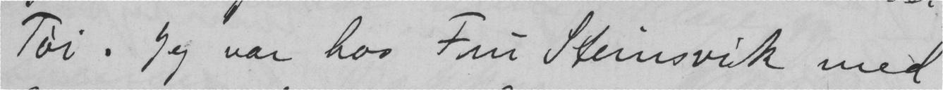Tøi. Jeg var hos Fru Steisvik med
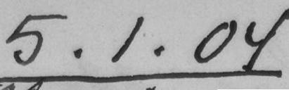5.1.04
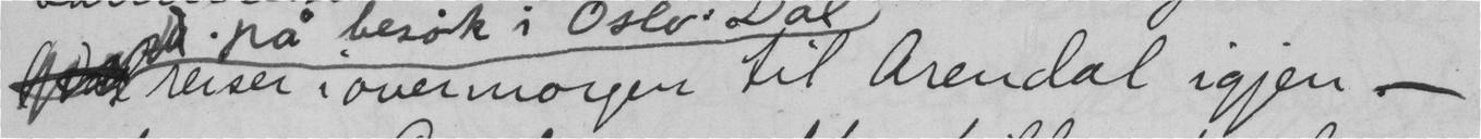reiser i overmorgen til Arendal igjen —
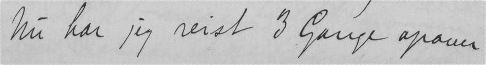Nu har jeg reist 3 Gange opover
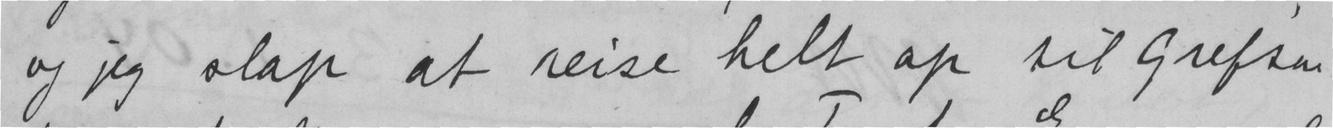og jeg slap at reise helt op til Grefsen
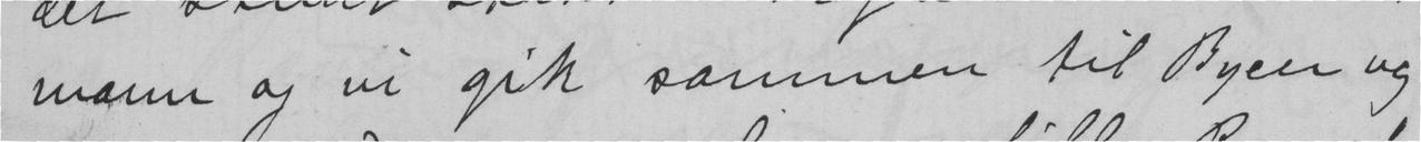mann og vi gik sammen til Byen og
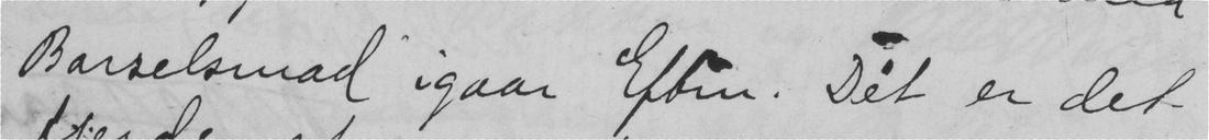Barselsmad igaar Eften. Det er det
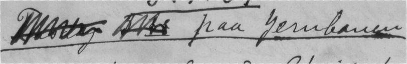paa Jernbanen
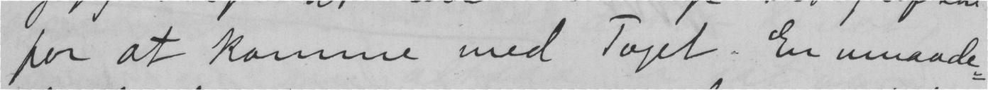for at komme med Toget. En umaade-
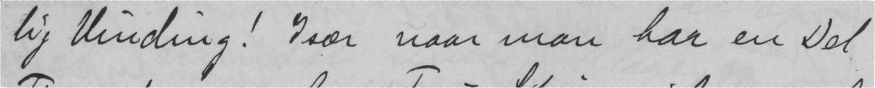lig Vinding! Især naar man har en Del
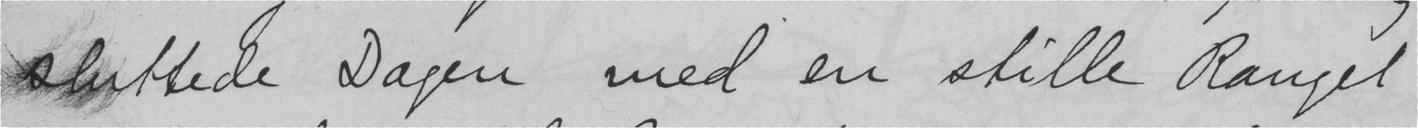sluttede Dagen med en stille Rangel
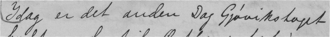Idag er det anden Dag Gjøvikstoget
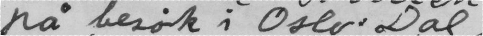på besøk i Oslo. Dal
